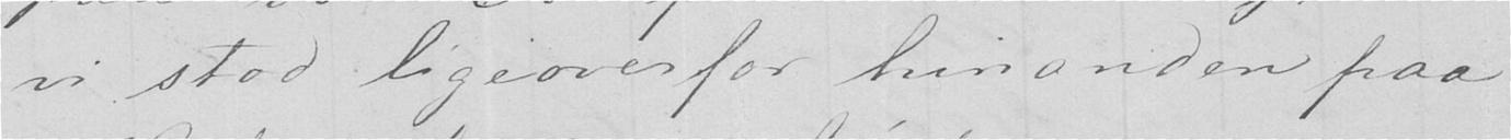vi stod ligeoverfor hinanden paa
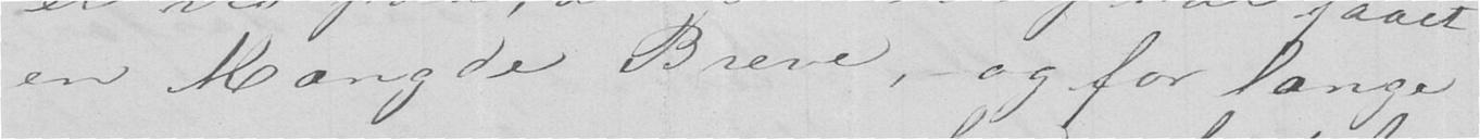en Mængde Breve, og for længe
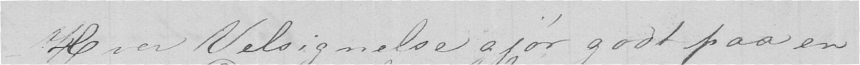- Hver Velsignelse gjør godt paa en
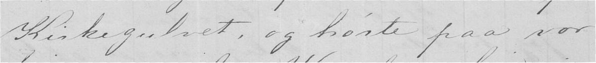Kirkegulvet, og hørte paa vor
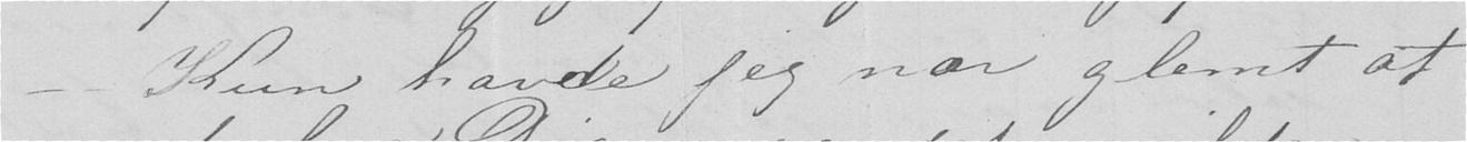- - Kun havde jeg nær glemt at
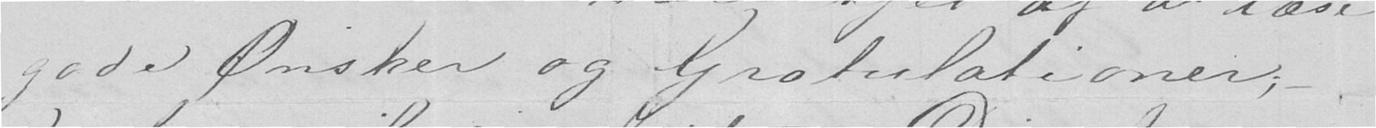gode Ønsker og Gratulationer; -
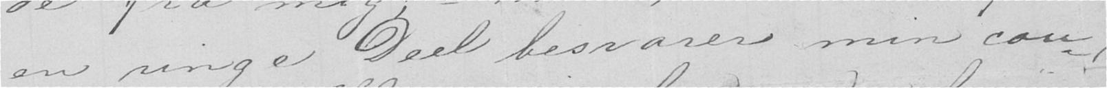en ringe Deel besvarer min cou-
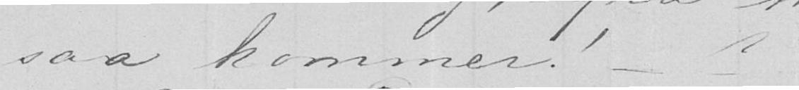saa kommer! - -
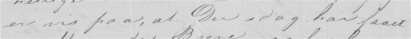er vis paa, at Du idag har faaet
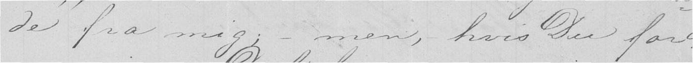de fra mig; - men, - hvis Du for
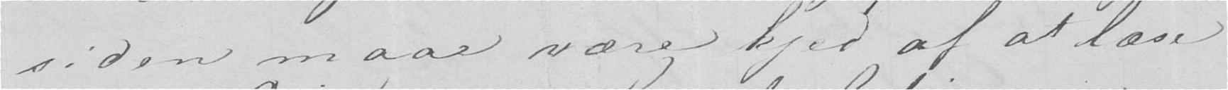siden maae være kjed af at læse
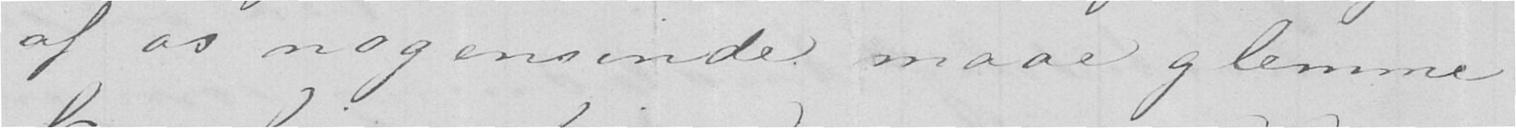af os nogensinde maae glemme
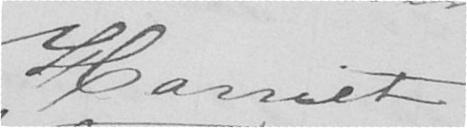Harriet
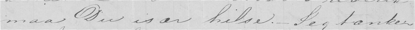maa Du især hilse. - Jeg tænker
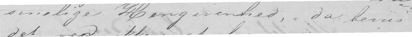sinelige Hengivenhed, da bevis
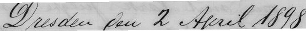Dresden den 2 April 1898
